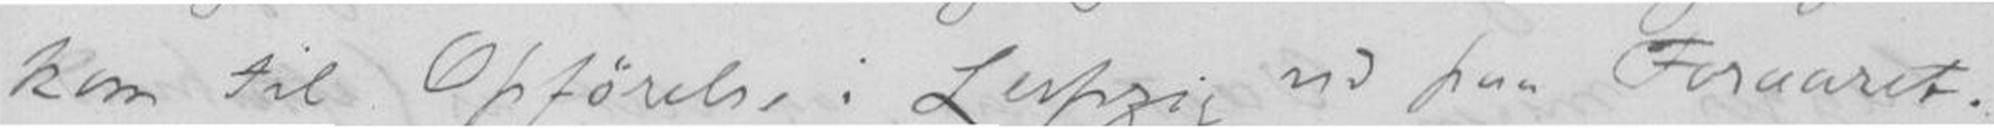kom til Opførelse i Leipzig ud paa Foraaret".
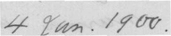4 Jan. 1900.
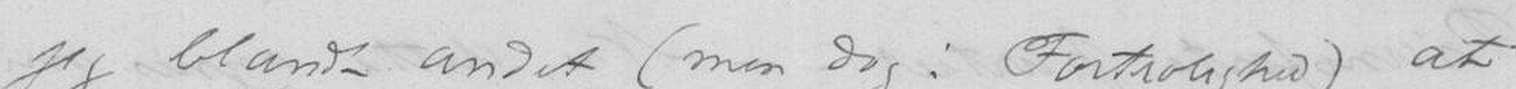jeg blandt andet (men dog: Fortrolighed) at
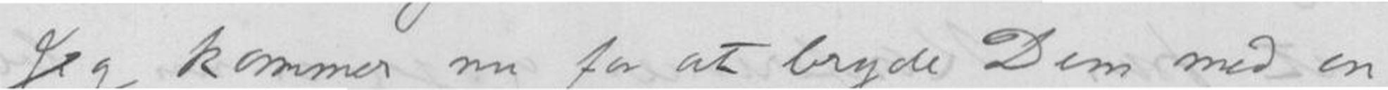Jeg kommer nu for at bryde Dem med en
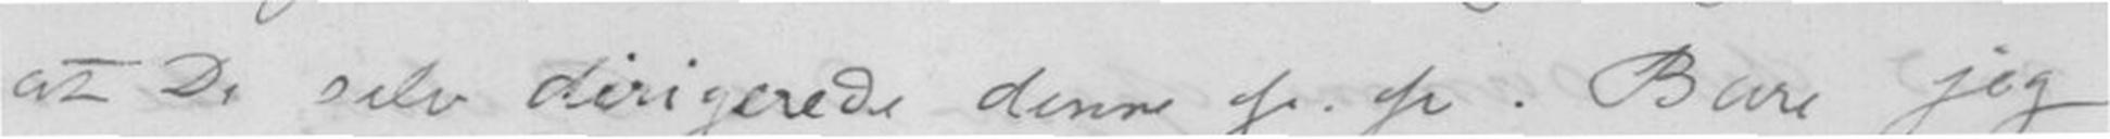at De selv dirigerede denne osv. osv. Bare jeg
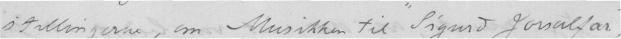stillingerne, om Musikken til "Sigurd Jorsalfar",
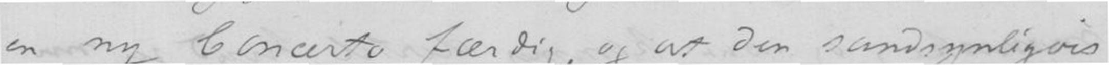en ny Concerto færdig, og at den sandsynligvis
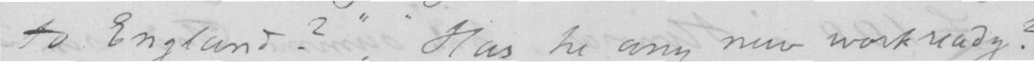to England?", "Has he any new work ready?
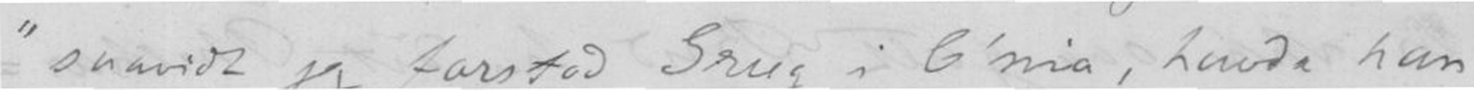"Saavidt jeg forstod Grieg i 'C'nia, havde han
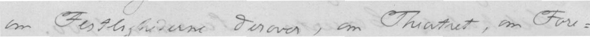om Festlighederne derover, om Theatret, om Fore-
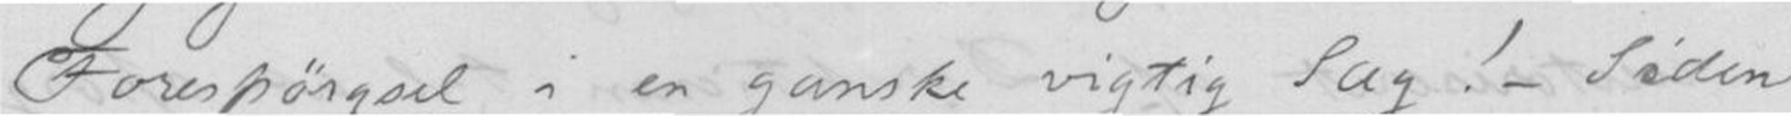Forespørgsel i en ganske vigtig Sag! - Siden
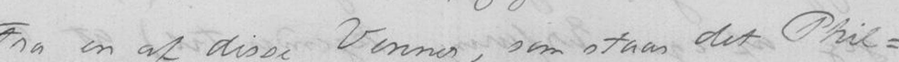Fra en af disse Venner, som staar det Phil-
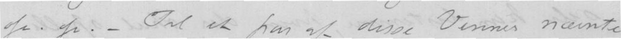osv. osv. - Til et par af disse Venner nævnte
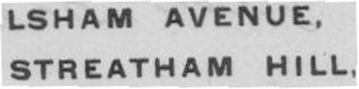STREATHAM HILL.
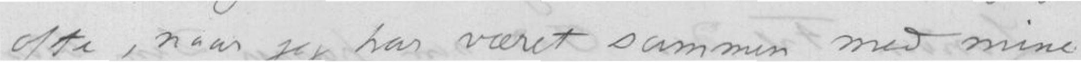ofte, naar jeg har været sammen med mine
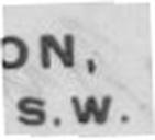S.W.
